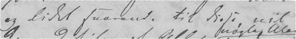og lidet svarende til disse, vil
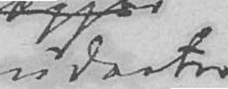udarter
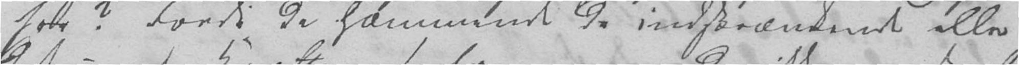for? Fordi de hæmmende de indskrænkende eller
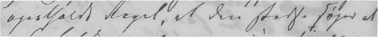opretholdt Regel, at den stedse søger at
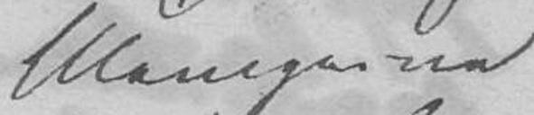Ulempene
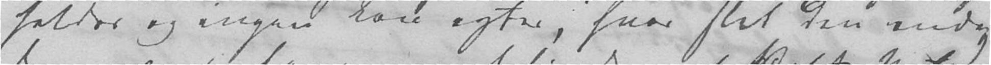holdes og ingen Lov agtes, hvor slet den enda
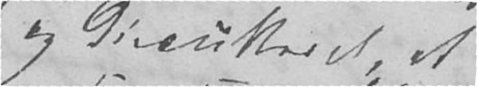og discuteret, at
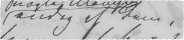endog af dem,
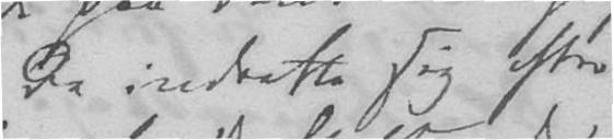De indrette sig efter
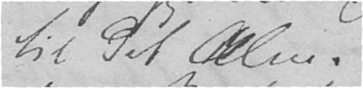til det Alm.
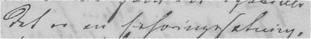Det er en Erfaringssætning,
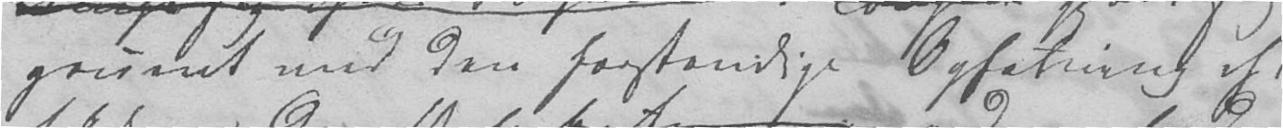gruent med den forstandige Opfatning af
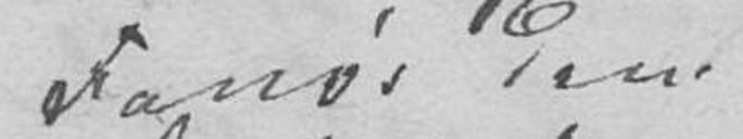Favør den
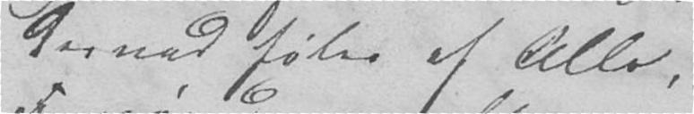derved føles af Alle,
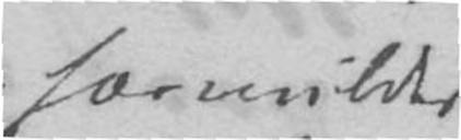formildes
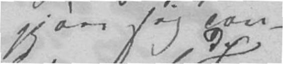gjøre sig con-
